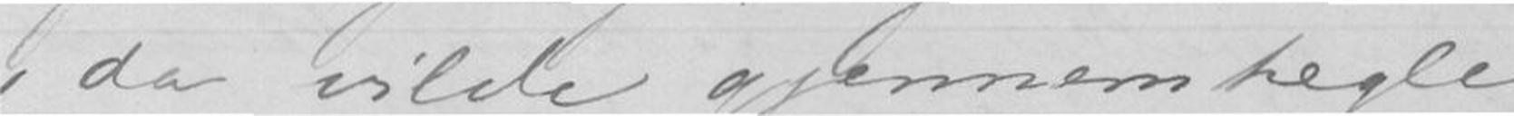da vilde gjennemhegle
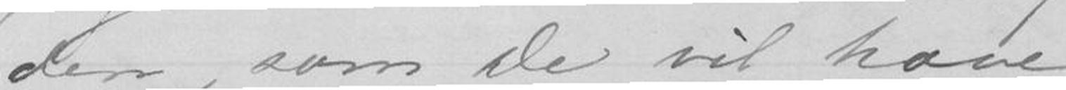den, som De vil have
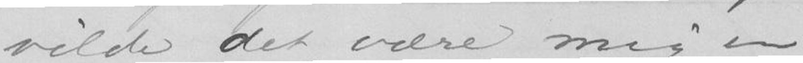vilde det være mig en
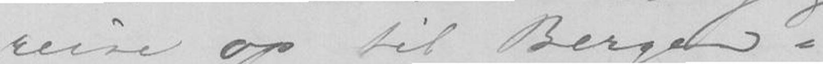reise op til Bergen i
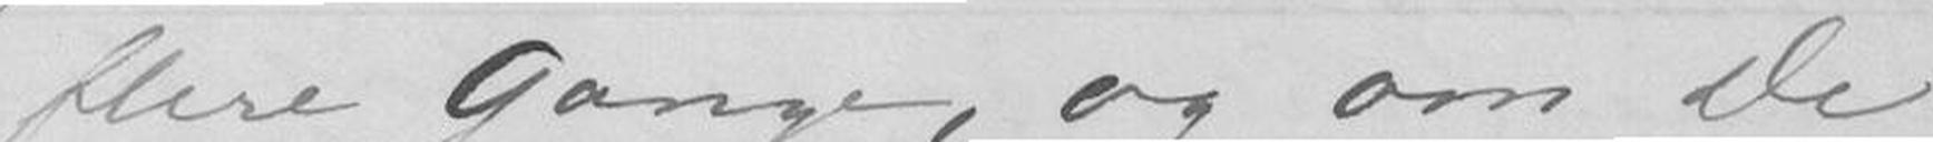flere Gange, og om De
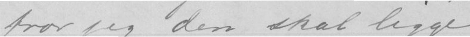tror jeg den skal ligge
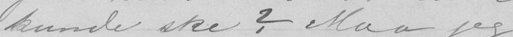kunde ske? Maa jeg
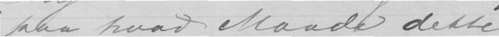paa hvad Maade dette
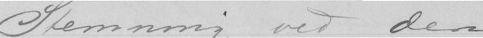Stemning ved den
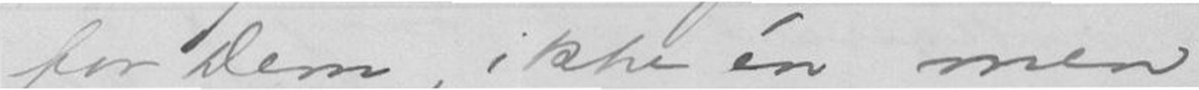for Dem, ikke én men
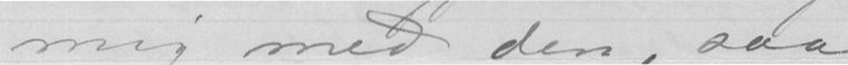mig med den, saa
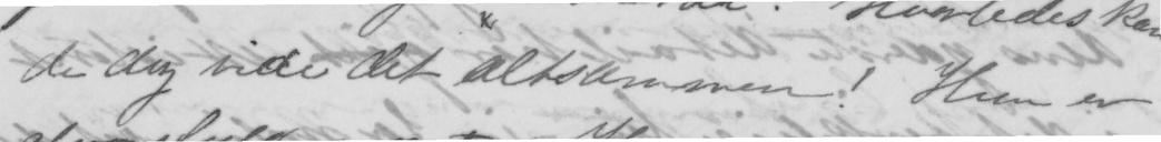de dog vide det altsammen! Hun er
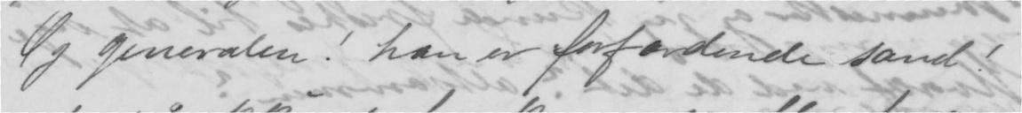Og generalen! han er forfærdende sand!
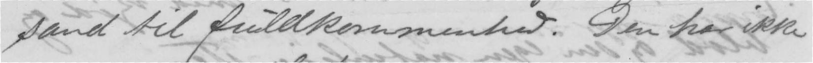sand til fuldkommenhed. Den har ikke
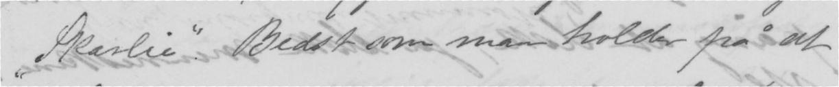"Skarlie". Bedst som man holder på at
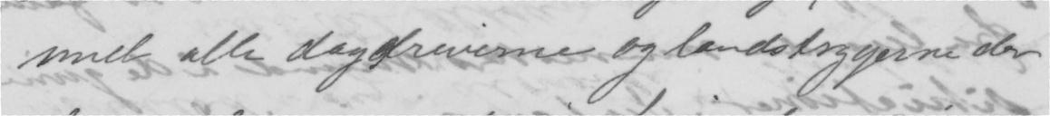lem alle dagdriverne og landstrygerne der
